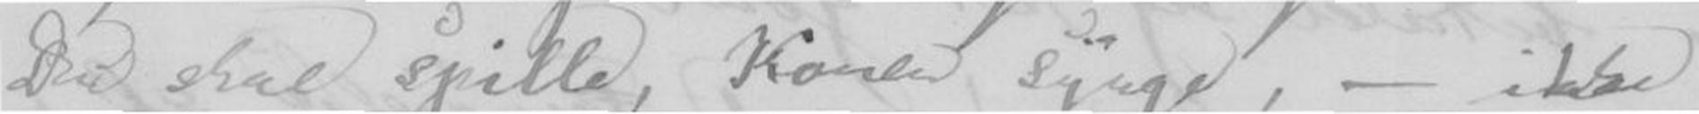Du skal spille, Konen synge, - ikke
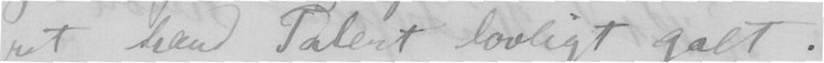ret hans Talent lovligt galt.
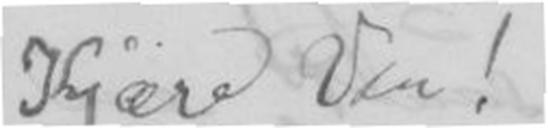Kjære Ven!
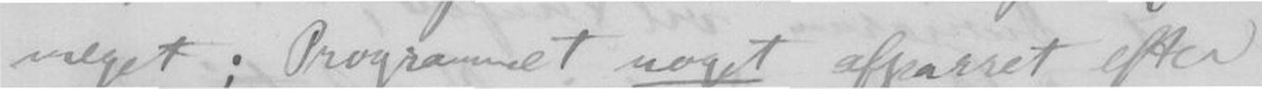meget; Programmet noget afpasset efter
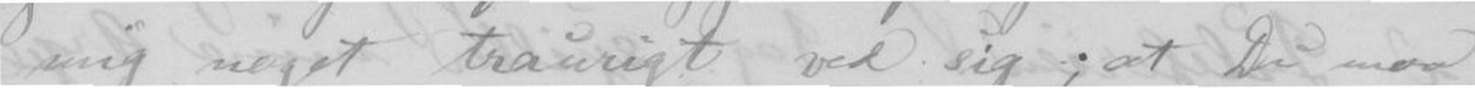mig meget traurigt ved sig; at Du maa
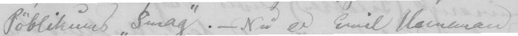Publikums Smag. - Nu er Emil Horneman
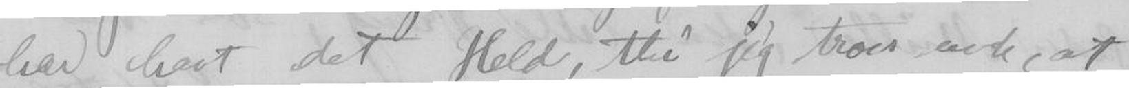har havt det Held, thi jeg troer nok, at
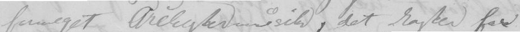formeget Orchestermusik, det koster for
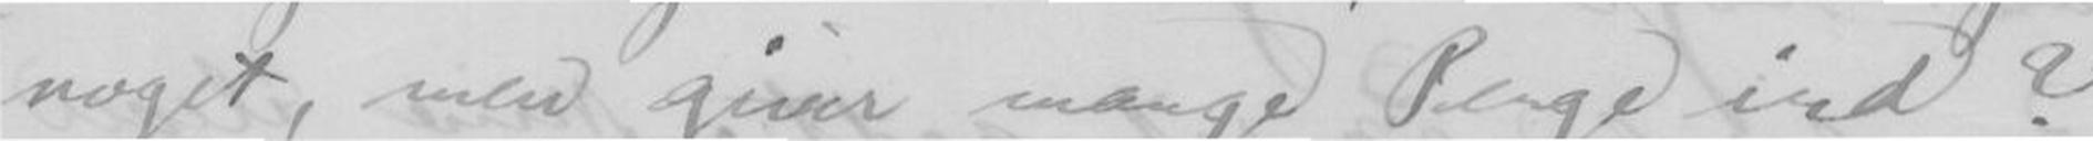noget, men giver mange Penge ind?
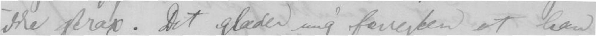ikke strax. Det glæder mig forresten at han
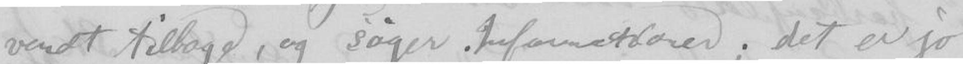vendet tilbage, og søger Informationer; det er jo
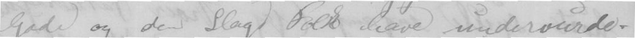Gade og den Slags Folk have undervurde-
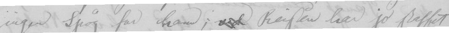ingen Spøg for ham; ved Reisen har jo skaffet
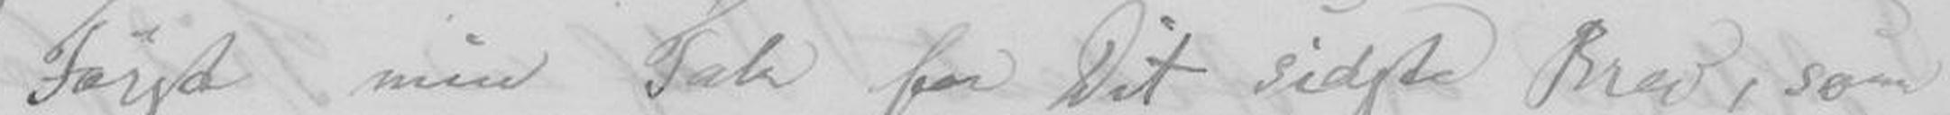Først min Tak for Dit sidste Brev, som
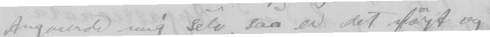Angaaende mig selv, saa er det først og
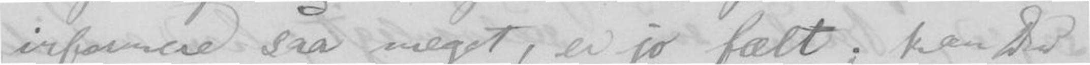informere saa meget; er jo fælt; kan Du
